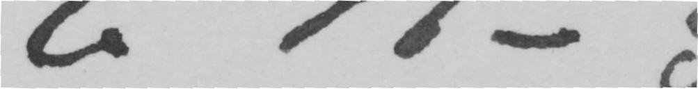E H-g.
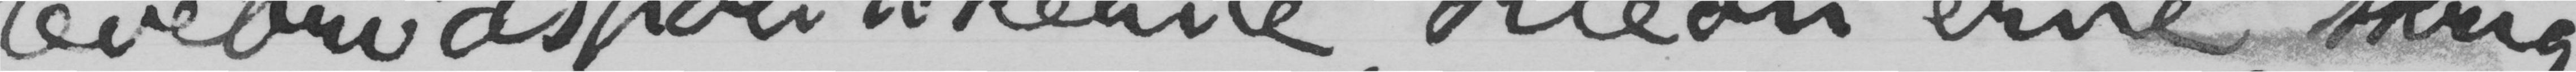levebrødspolitikerne, Kleon’erne, skrig-
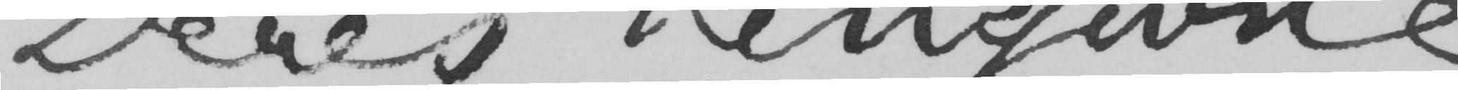Deres hengivne
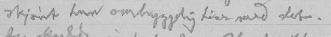skjønt han omhyggelig tier med det.
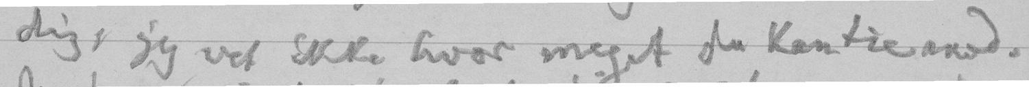dig, jeg vet ikke hvor meget du kan tie med.
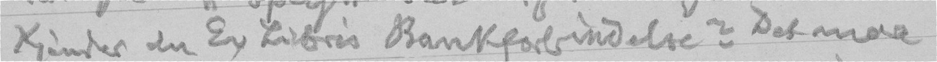Kjender du Ex Libris Bankforbindelse? Det maa
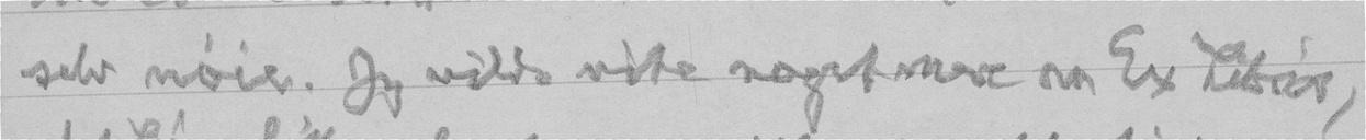selv nøie. Jeg vilde vite noget mere om Ex Libris,
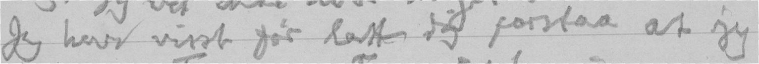Jeg har visst før latt dig forstaa at jeg
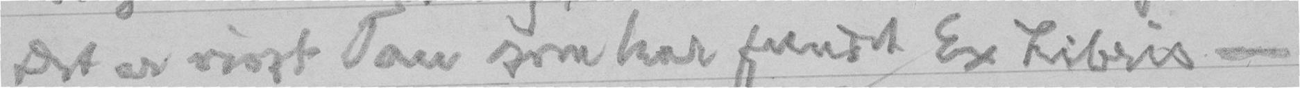Det er visst Tau som har fundet Ex Libris -
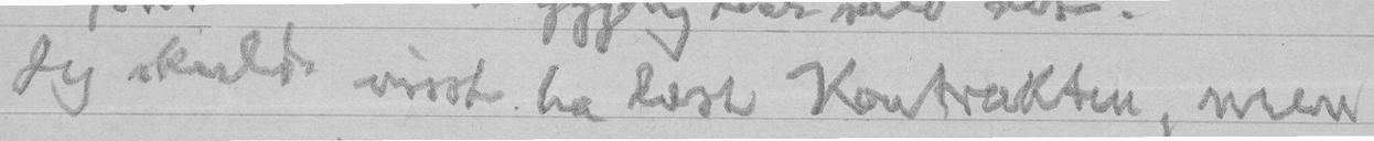Jeg skulde visst ha læst Kontrakten, men
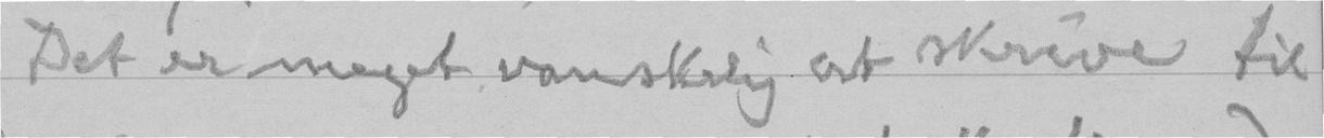Det er meget vanskelig at skrive til
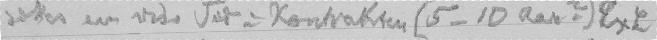sættes en vid Tid i Kontrakten (5-10 Aar?) Ex L
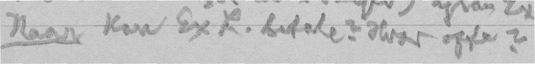Naar kan Ex L. betale? Hvor ofte?
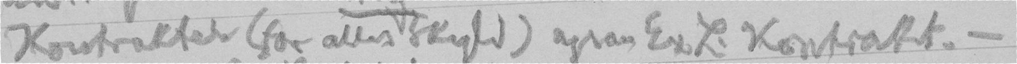Kontrakter (for alles Skyld) ogsaa Ex L. Kontrakt. -
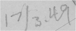17/3. 49
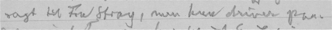sagt til Fru Stray, men hun driver paa.
